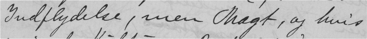Indflydelse, men Magt, og hvis
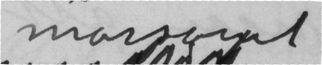morsomt
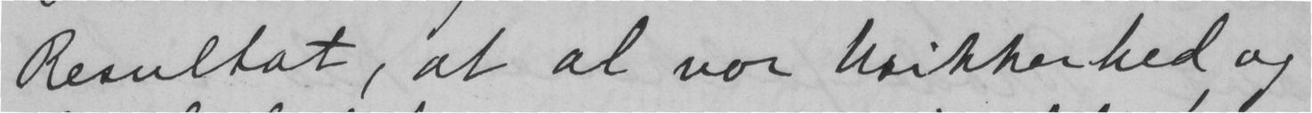Resultat, at al vor usikkerhed og
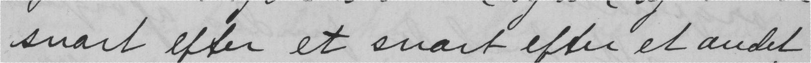snart efter et snart efter et andet
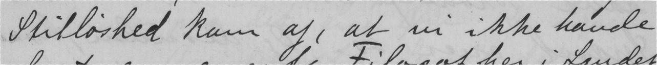Stilløshed kom af, at vi ikke havde
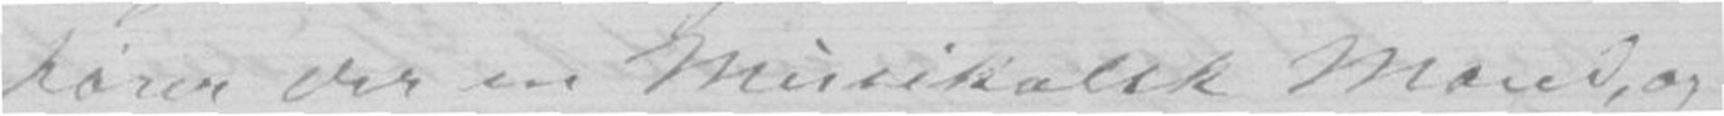hører der en Musikalsk Mand, og
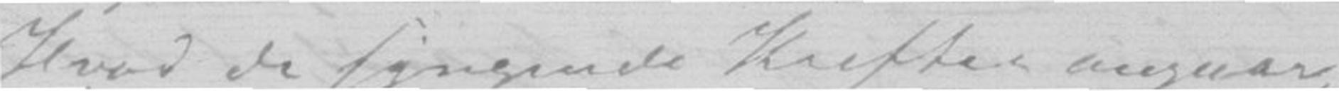Hvad de syngende Krefter angaar,
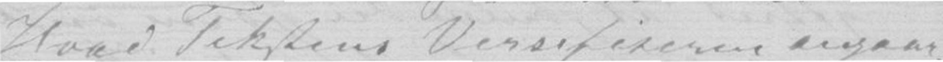Hvad Tekstens Versefiteren angaar,
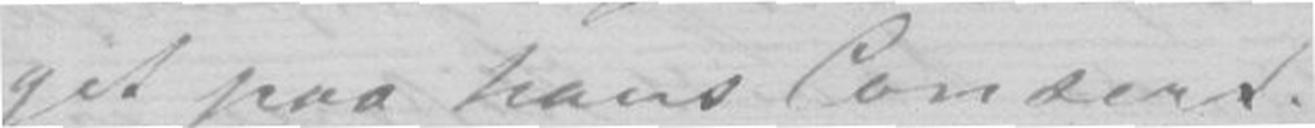get paa hans Consert.
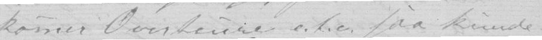komer Overtcure e.t.c. saa kunde
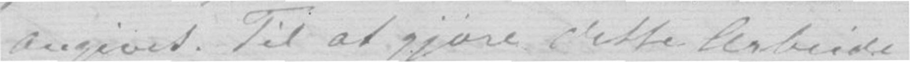angivet. Til at gjøre dette Arbeide
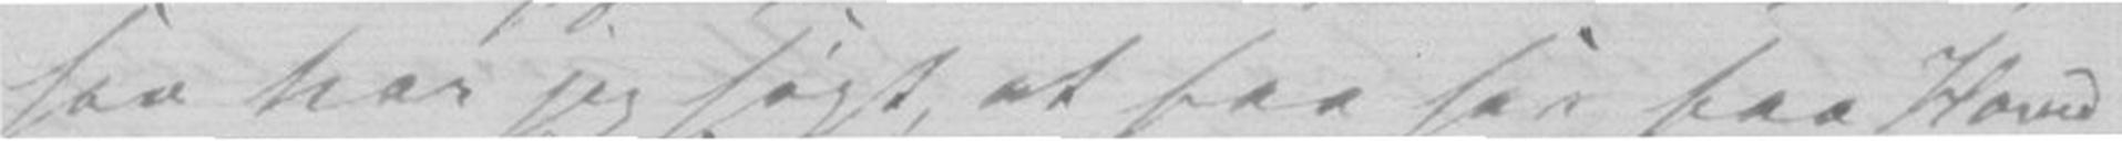saa har jeg søgt, at faa saa faa Hoved
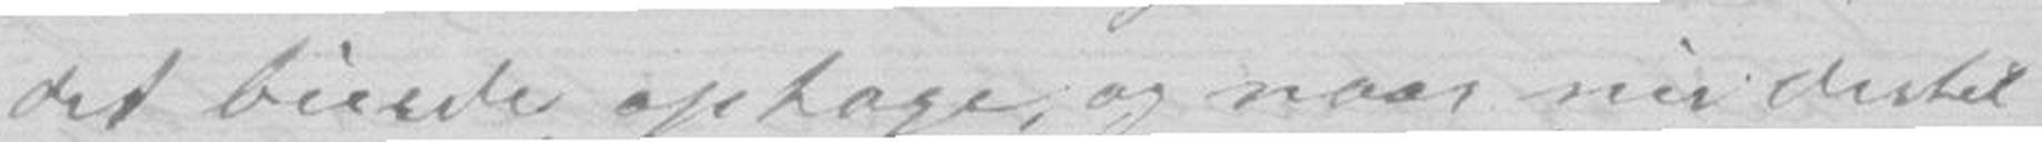det burde optage, og naar nu dertil
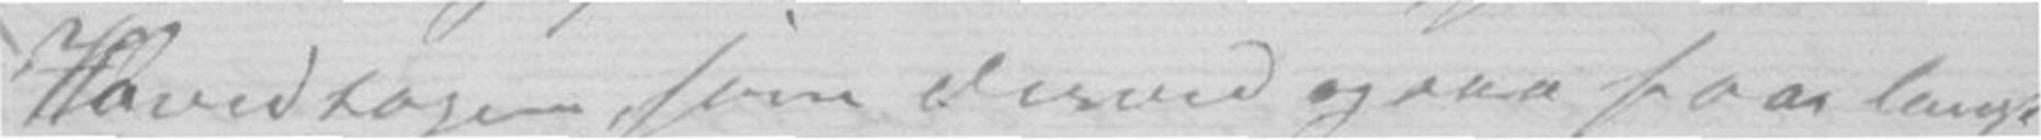Hovedsagen som derved ogsaa faar langt
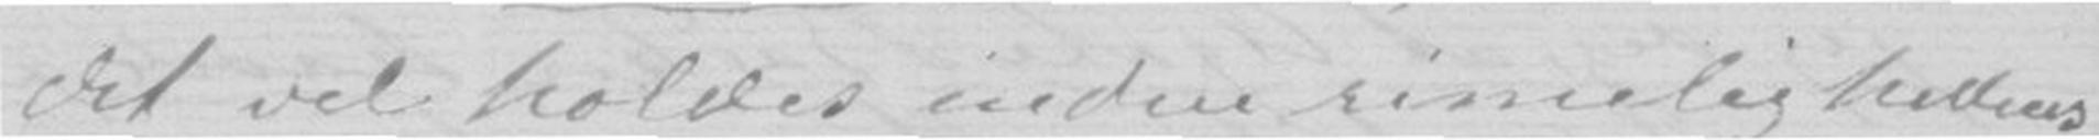det vel holdes inden rimelighedens
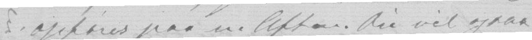opføres paa en Aften. Du vil ogsaa
